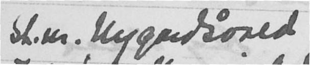St.m. Nygårdsvold
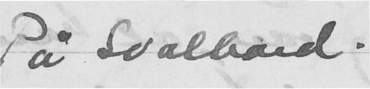På Svalbard.
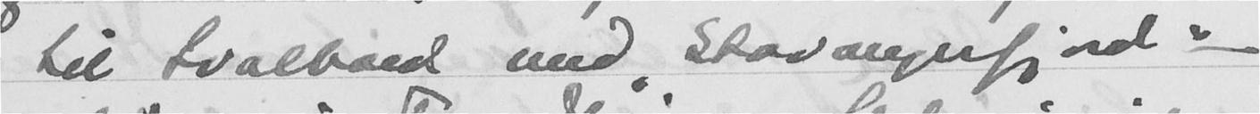til Svalbard med "Stavangerfjord" -
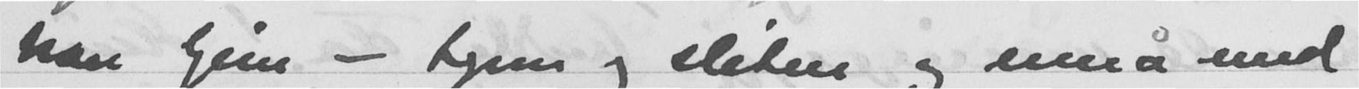han hjem - hjem og sliten og ennå med
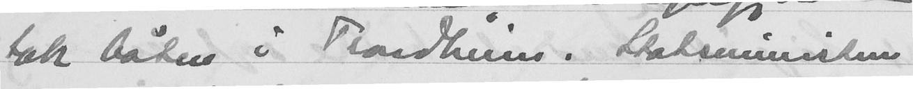tok båten i Trondheim. Statsministeren
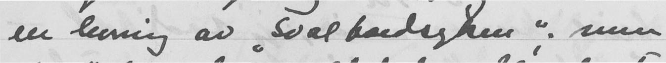en levning av "svalbardsyken", men
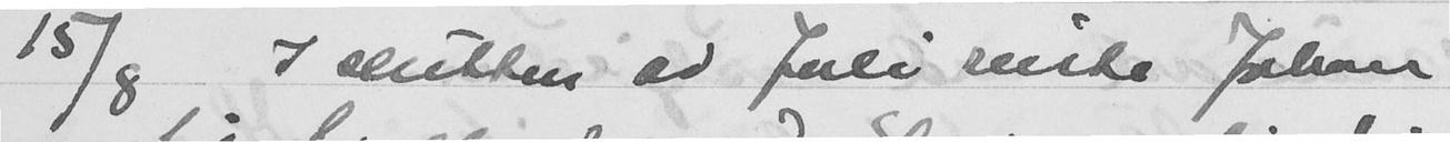15/8 I slutten av Juli reiste Johan
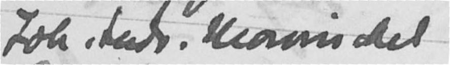Joh. Lud. Mowinckel
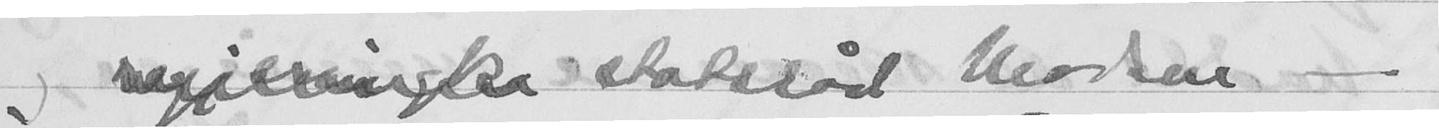og nyyerunglea statsråd Madsen -
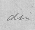din
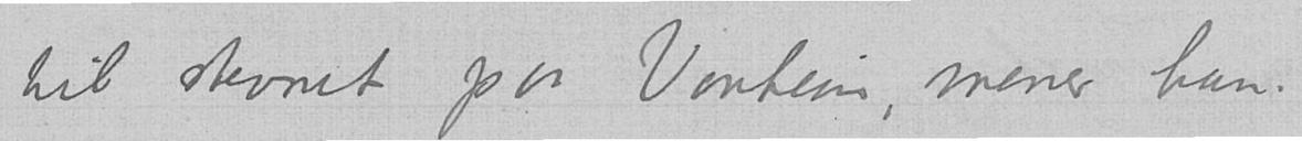til stevnet paa Vonheim, mener han.
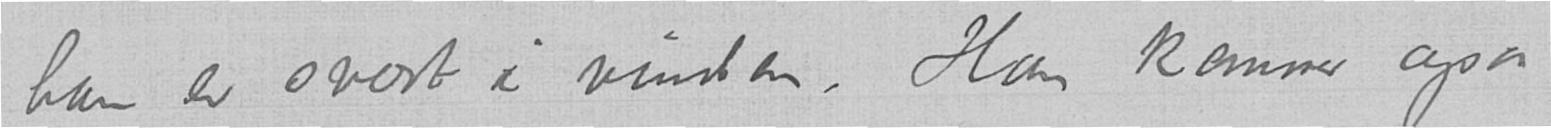han er svært i vinden. Han kommer ogsa
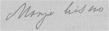Mange hilsener
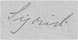Sigrid.
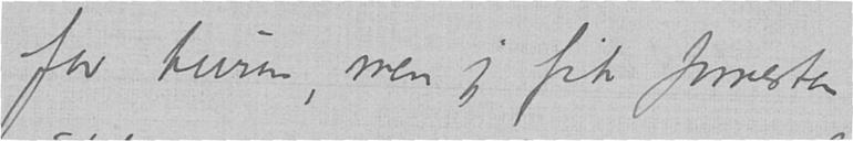for turen, men jeg fik forresten
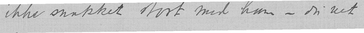ikke snakket stort med ham - du vet
